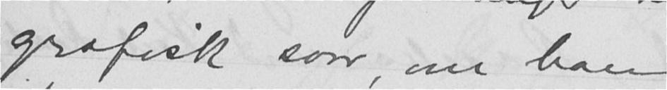grafisk svar om han
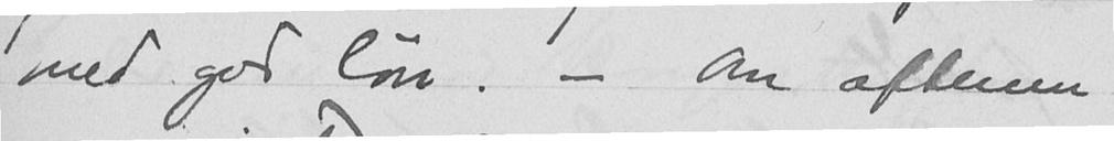med god løn. - Om aftenen
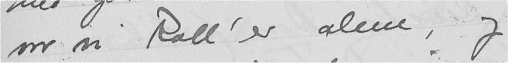var vi Roll'er alene, og
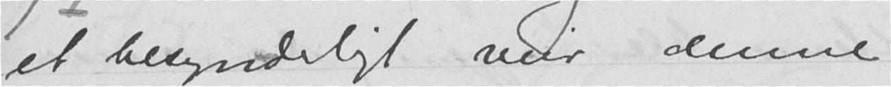et besynderligt veir denne
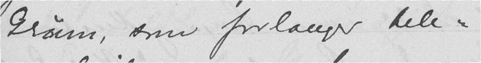Grønn, som forlanger tele-
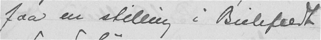faa en stilling i Bielefeldt
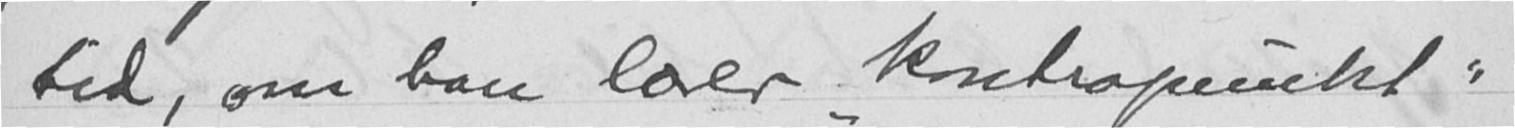tid, om han lærer "kontrapunkt"
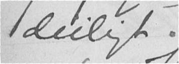deiligt.
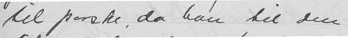til paaske, da han til den
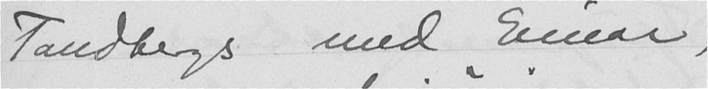Tandbergs med Einar,
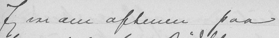Jeg var om aftenen paa
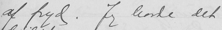af fryd. Jeg havde det
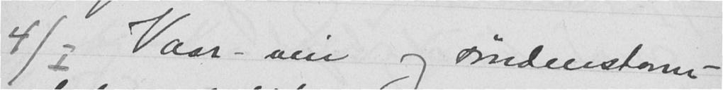4/I Vaar-veir og søndenstorm -
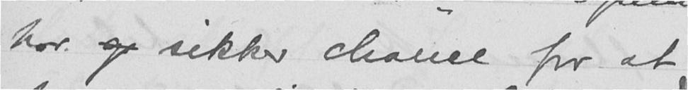har op sikker chance for at
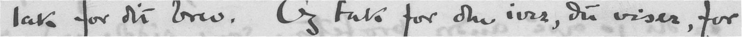Tak for dit brev. Og tak for den iver, du viser, for
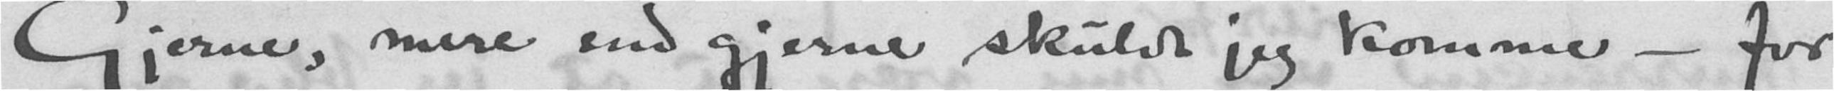Gjerne, mere end gjerne skulde jeg komme - for
